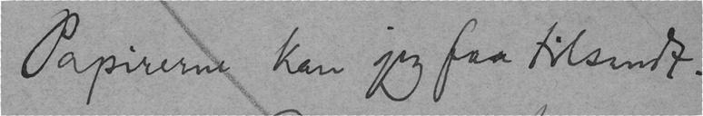Papirerne kan jeg faa tilsendt.
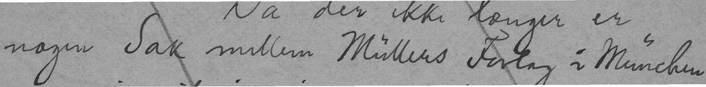nogen Sak mellem Müllers Forlag i München
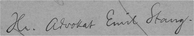Hr. Advokat Emil Stang.
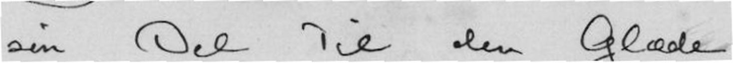sin Del til den Glæde
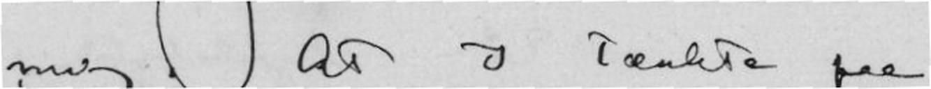mig. At I tænkte paa
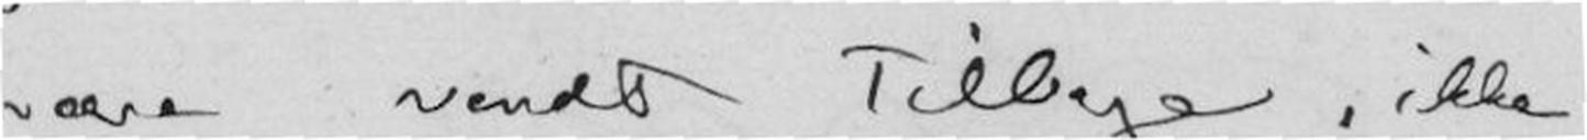være vendt Tilbage, ikke
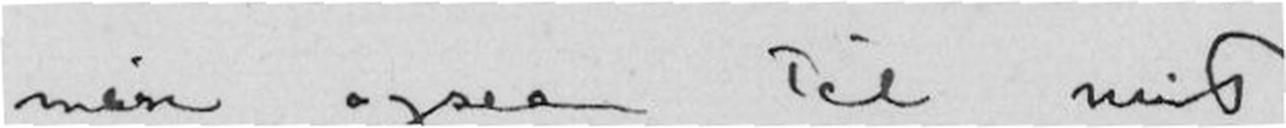men ogsaa til mit
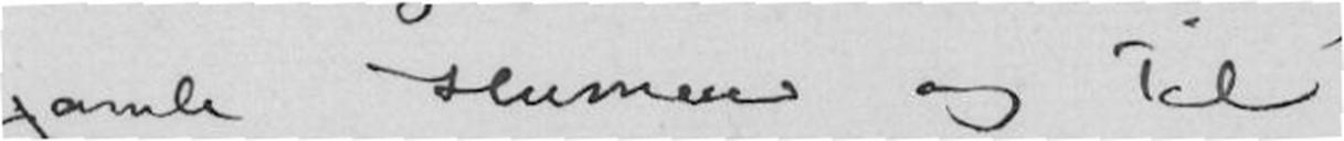gamle Humeur og til
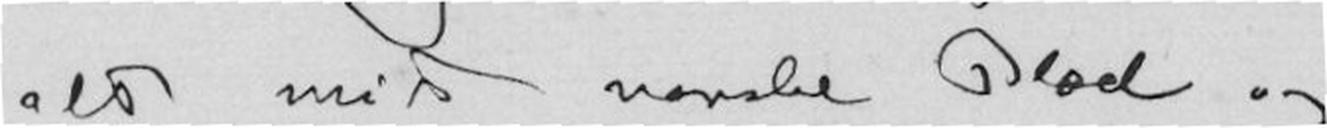alt mit norske Blod og
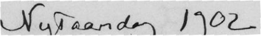Nytaarsdag 1902.
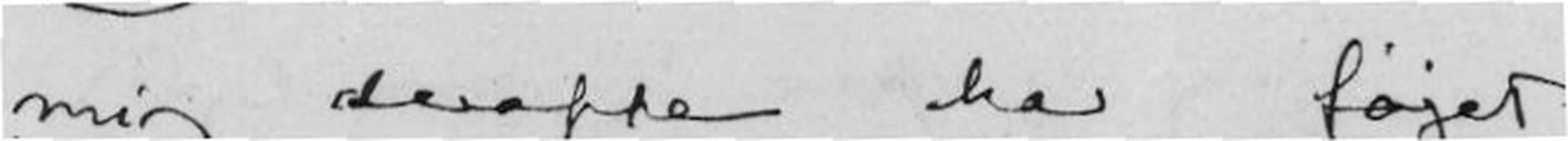mig deroppe har føjet
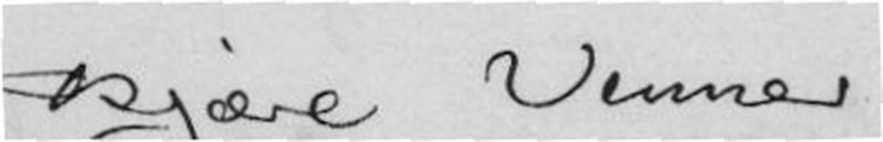Kjære Venner
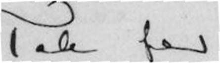Tak for
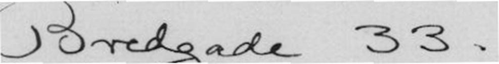Bredgade 33.
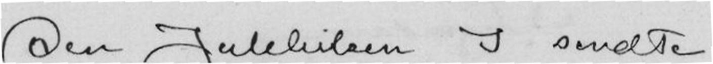Den Julehilsen I sendte
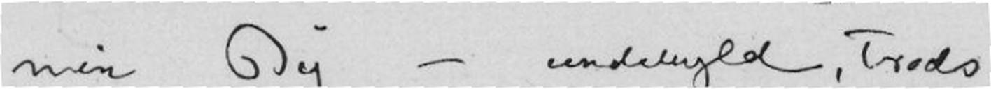min By - undskyld, Trods
